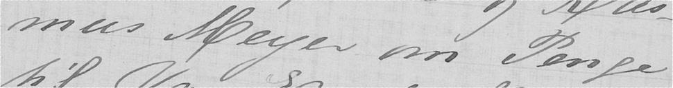mus Meyer om Penge
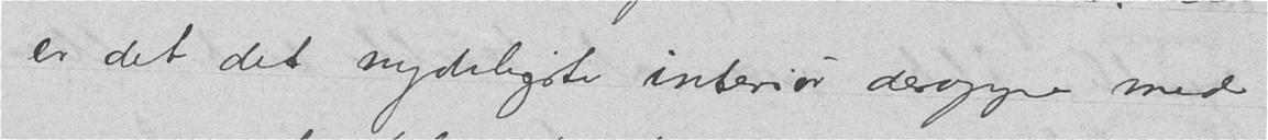er det det nydeligste interiør deroppe med
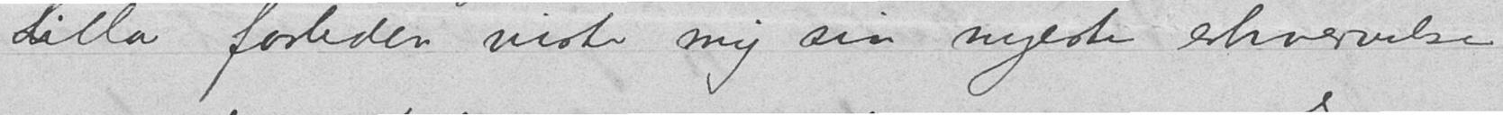Lilla forleden viste mig sin nyeste ehvervelse
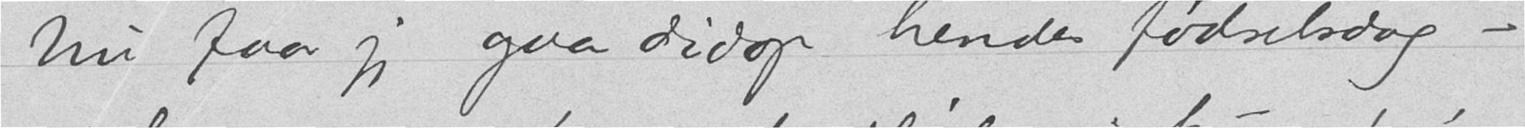Nu faar jeg gaa didop hendes fødselsdag –
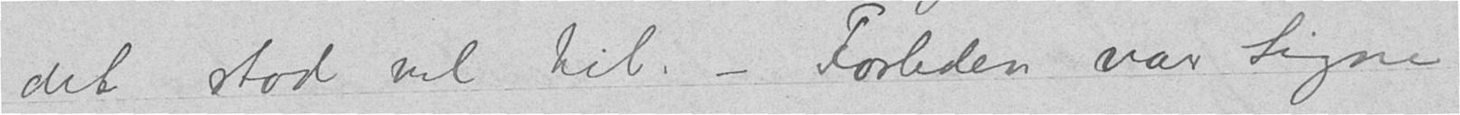det stod vel til. – Forleden var Signe
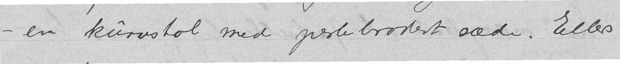– en kurvstol med perlebrodert sæde. Ellers
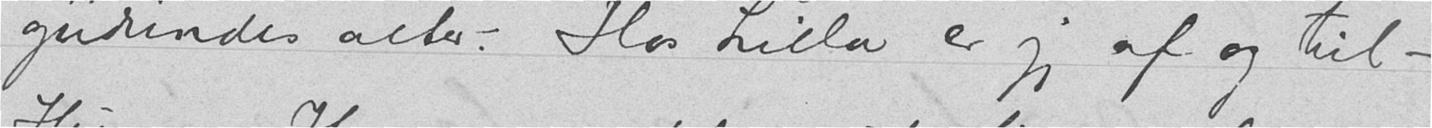gudindes alter. Hos Lilla er jeg af og til –
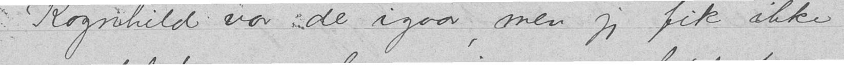Ragnhild var ude igaar, men jeg fik ikke
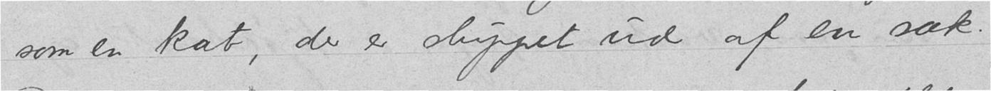som en kat, der er sluppet ud af en sæk.
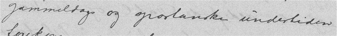gammeldags og spartanske undertiden
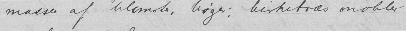masser af blomster, bøger –, birketræs møbler –
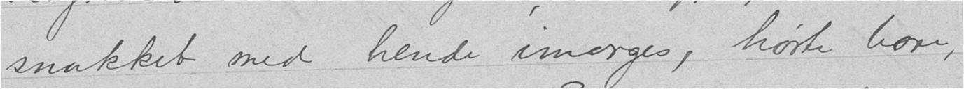snakket med hende imorges, hørte bare,
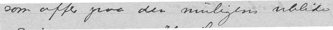som offer paa den muligens ublide
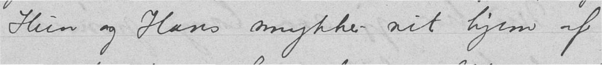Hun og Hans smykker sit hjem af
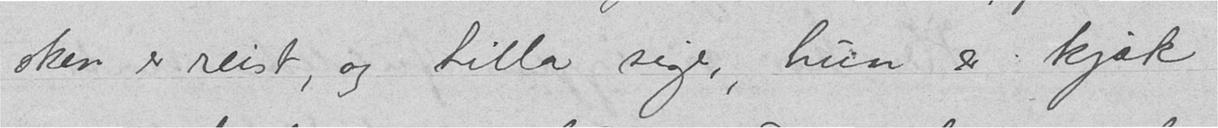sken er reist, og Lilla siger, hun er kjæk
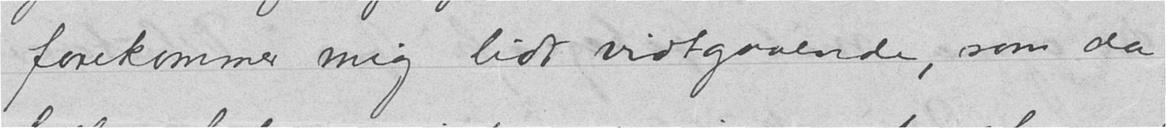forekommer mig lidt vidtgaaende, som da
